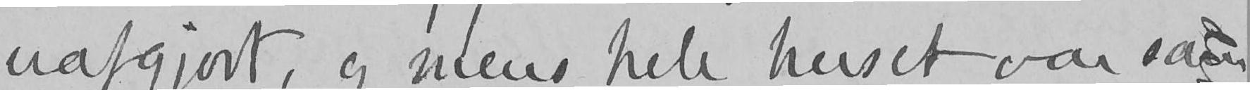uafgjort, og mens hele huset var sam-
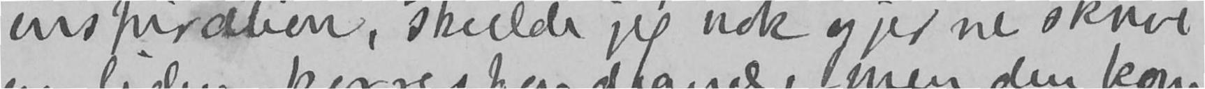inspiration, skulde jeg nok gjerne skrive
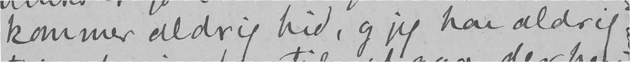kommer aldrig hid, og jeg har aldrig
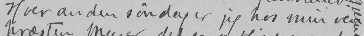Hver anden søndag er jeg hos min ven
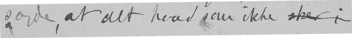sagde, at alt hvad som ikke sker i
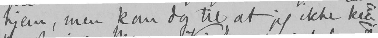hjem, men kom dog til, at jeg ikke kun-
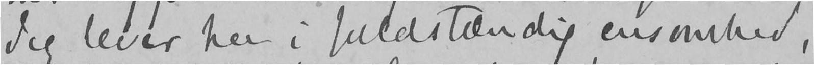Jeg lever her i fuldstændig ensomhed,
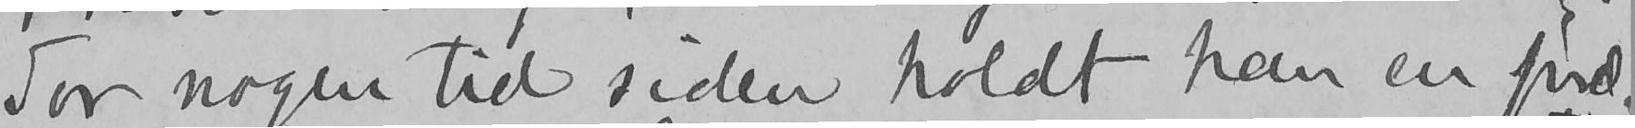For nogen tid siden holdt han en præ-
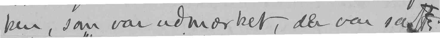ken, som var udmærket, der var saft
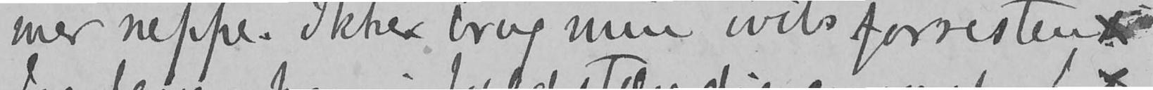mer neppe. Ikke brug min vits forresten x
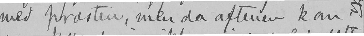med præsten, men da aftenen kom
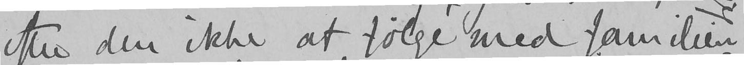efter den ikke at følge med familien
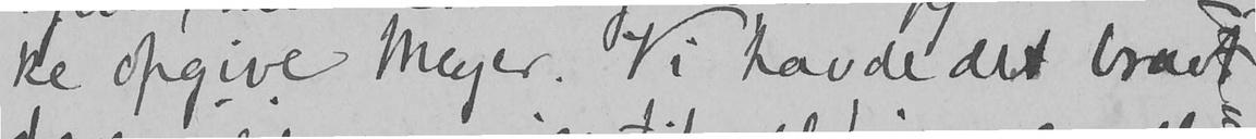ke opgive Meyer. Vi havde det bravt
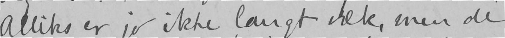Alliks er jo ikke langt væk, men de
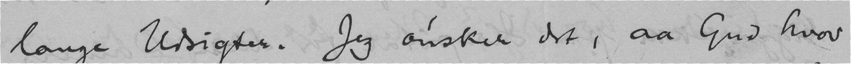lange Udsigter. Jeg ønsker det, aa Gud hvor
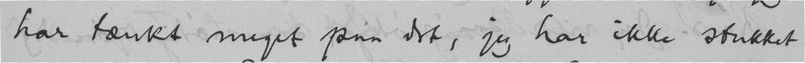har tænkt meget paa det, jeg har ikke stukket
